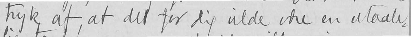tryk af, at det for dig vilde være en utaale-
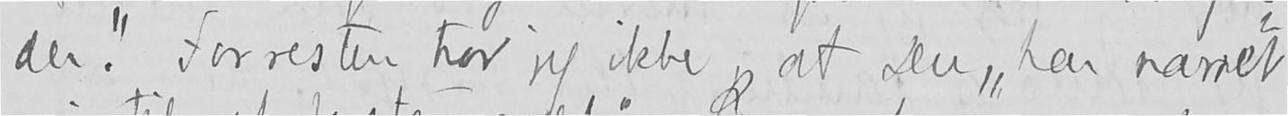der. Forresten tror jeg ikke, at Du "har narret
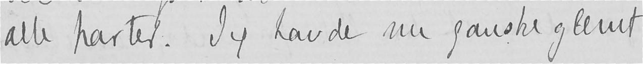alle parter. Jeg havde nu ganske glemt
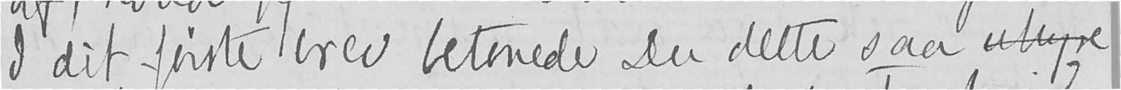I dit første brev, betonede Du dette saa uhyre
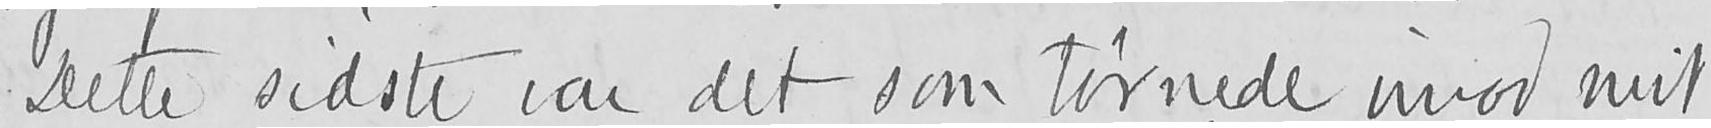Dette sidste var det som tørnede imod mit
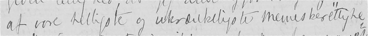af vore helligste og ukrænkeligste menneskerettighe-
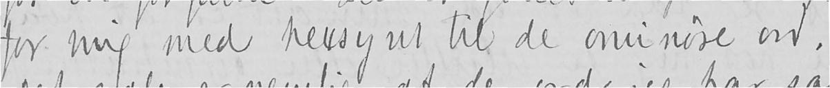for mig med hensyn til de ominøse ord.
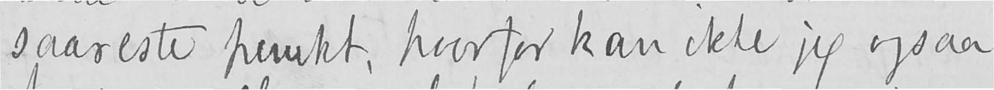saareste punkt, hvorfor kan ikke jeg ogsaa
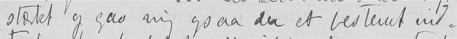stærkt og gav mig ogsaa der et bestemt ind-
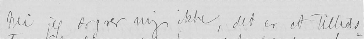Nei jeg ærgrer mig ikke, det er et tillids-
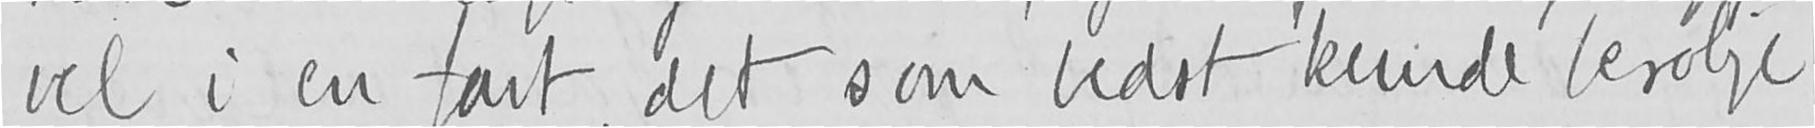vel i en fart det som bedst kunde berolige
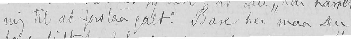mig til at forstaa galt". Bare her maa Du
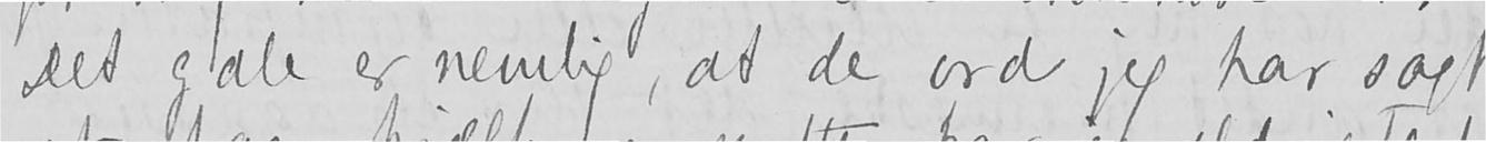Det gale er nemlig, at de ord jeg har sagt
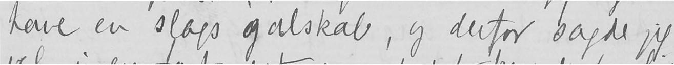have en slags galskab, og derfor sagde jeg
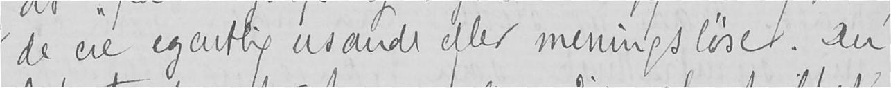de ere egentlig usande eller meningsløse. Du
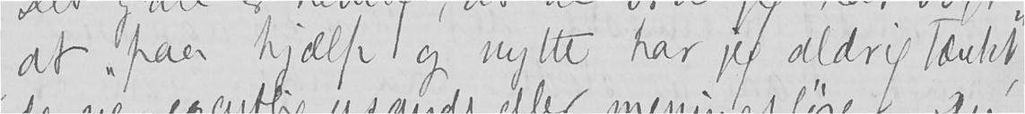at "paa hjælp og nytte har jeg aldrig tænkt"
